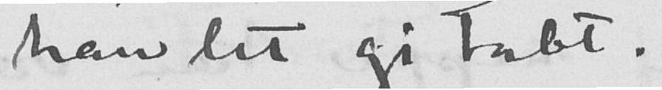han let gi tabt.
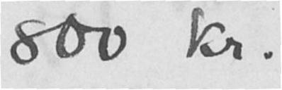800 kr.
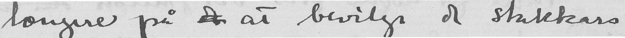længere på de at bevilge de stakkars
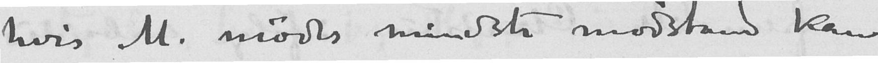hvis M. møder mindste modstand kan
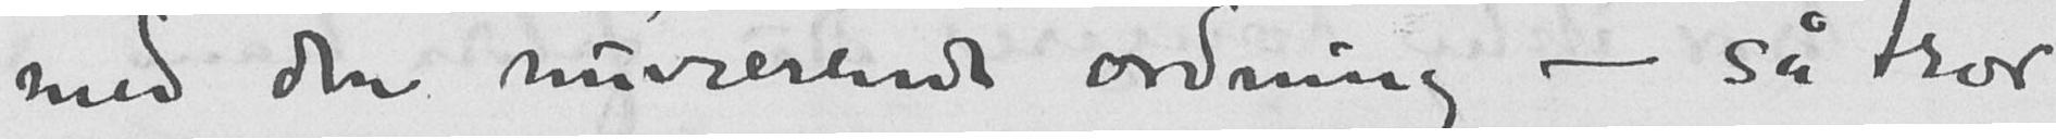med den nuværende ordning - så tror
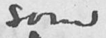som
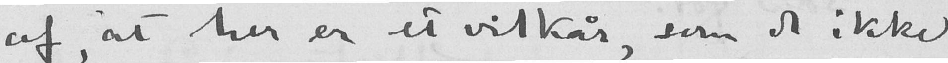af, at her er et vilkår, som de ikke
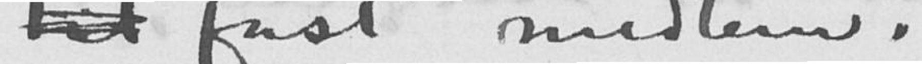til fast medlem.
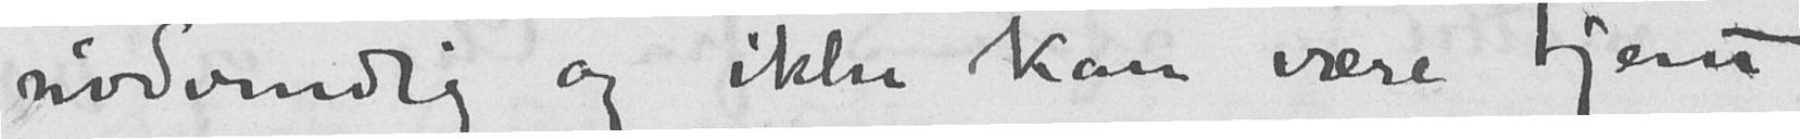nødvendig og ikke kan være tjent
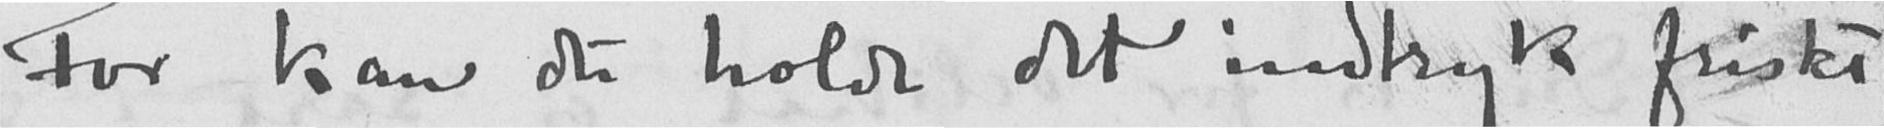For kan du holde det indtryk friskt
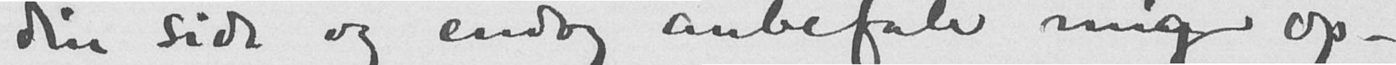din side og endog anbefale mig op-
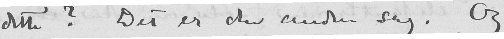dette? Det er den anden sag. Og
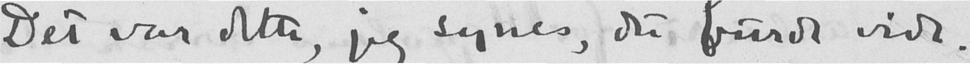Det var dette, jeg synes, du burde vide.
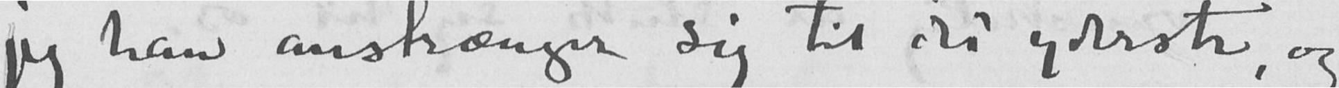jeg han anstrænger sig til det yderste, og
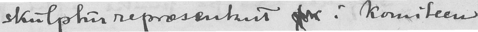skulpturrepræsentant for i komiteen
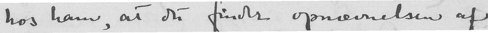hos ham, at du finder opnævnelsen af
